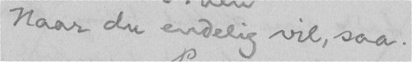Naar du endelig vil, saa.
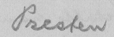Presten
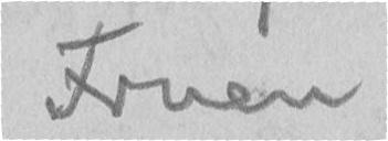Fruen
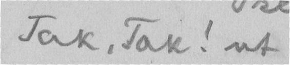Tak, Tak! ut
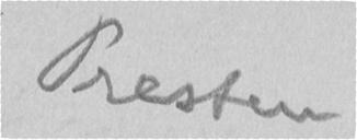Presten
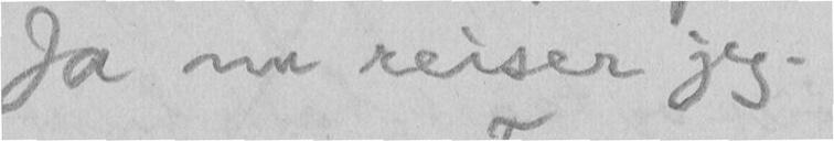Ja nu reiser jeg.
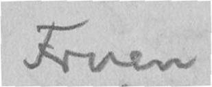Fruen
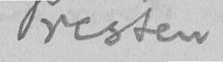Presten
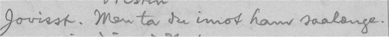Jovisst. Men ta du imot ham saalænge.
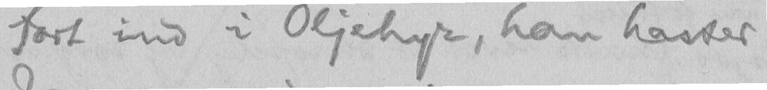fort ind i Oljehyr, han haster
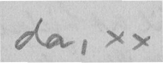da, x x
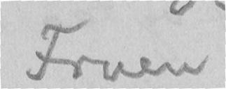Fruen
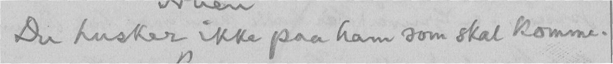Du husker ikke paa ham som skal komme.
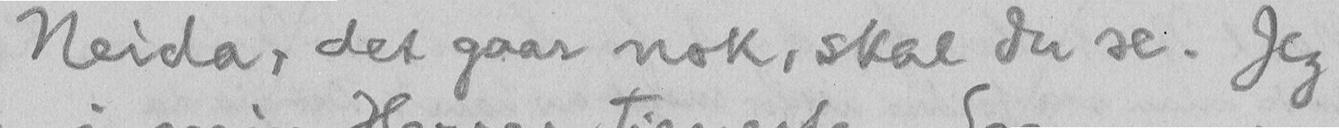Neida, det gaar nok, skal du se. Jeg
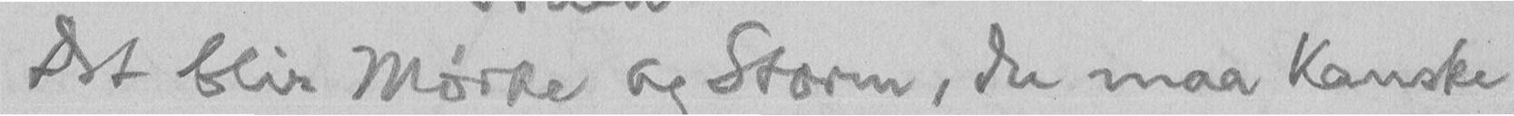Det blir Mørke og Storm, du maa kanske
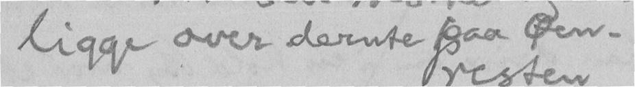ligge over derute paa Øen.
